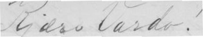Kjære Vardo!
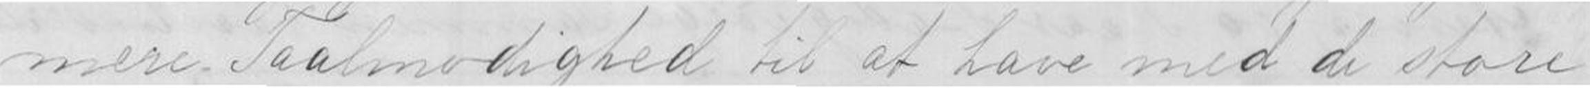mere Taalmodighed til at have med de store
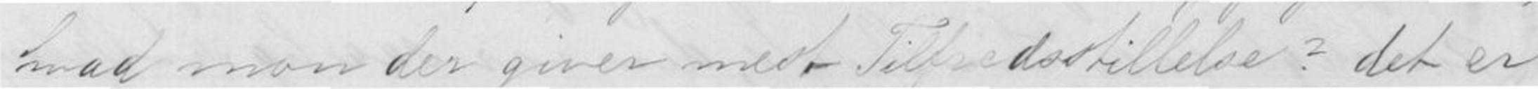hvad mon der giver mest Tilfredsstillelse? det er
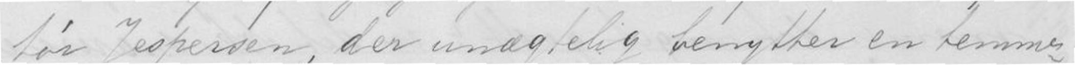tør Jespersen, der unægtelig benytter en temme-
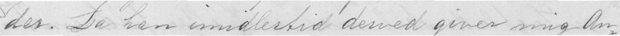der. Da han imidlertid dermed giver mig An-
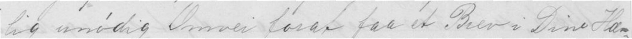lig unødig Omvei forat faa et Brev i Dine Hæn-
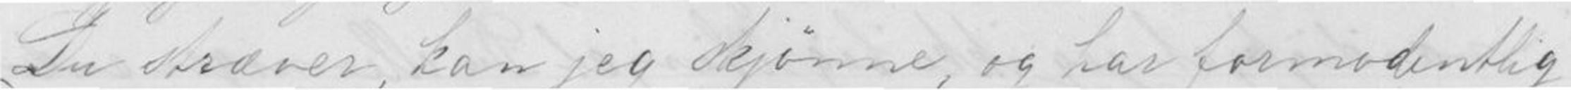Du stræver, kan jeg skjønne, og har formodenlig
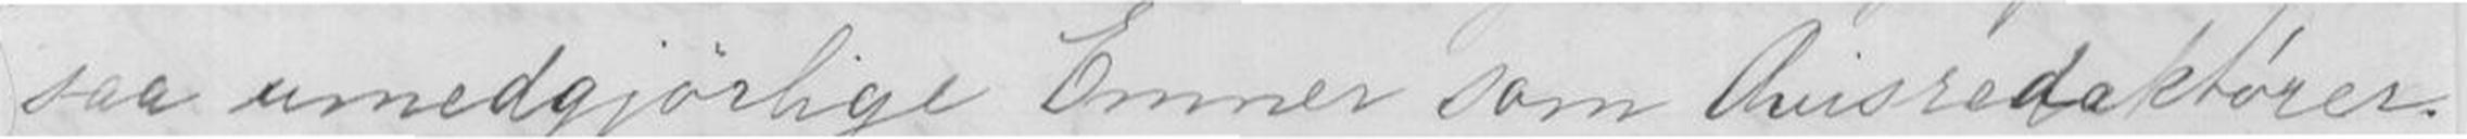saa umedgjørlige Emner som Avisredaktører.
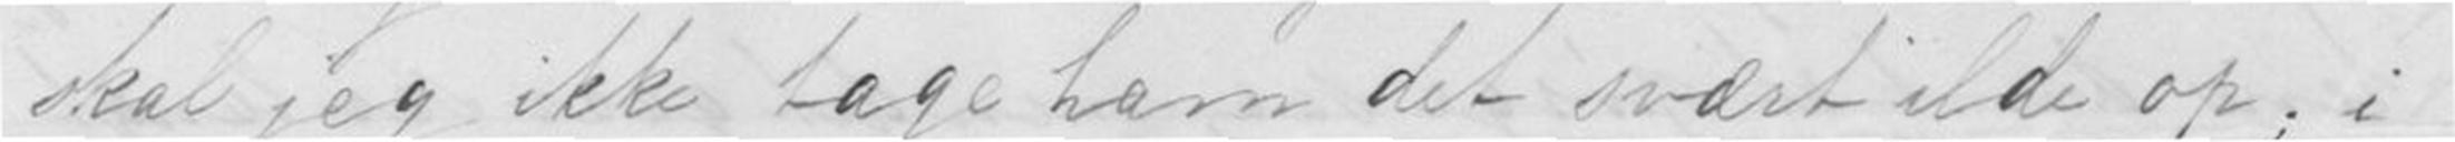skal jeg ikke tage ham det svært ilde op; i
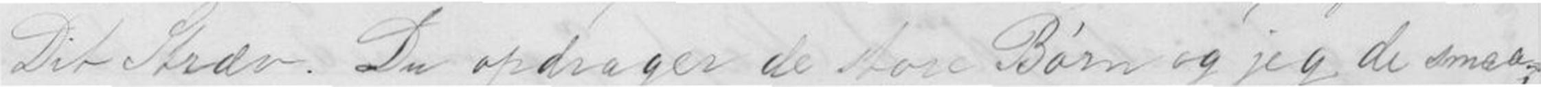Dit Stræv. Du opdrager de store Børn og jeg de smaa,
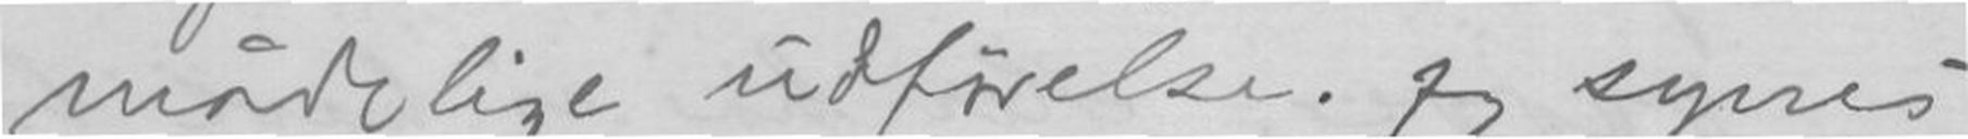mådelige udførelse. Jeg synes
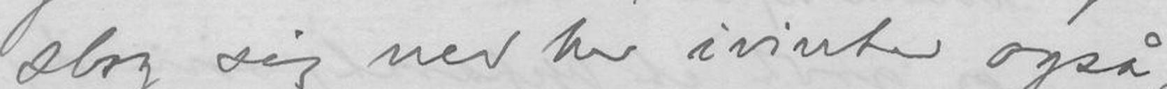slog sig ned her ivinter også,
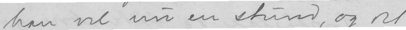han vel nu en stund, og det
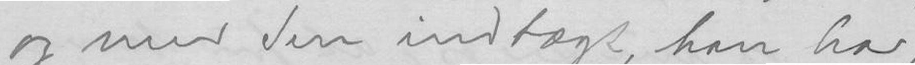og med den intægt, han har,
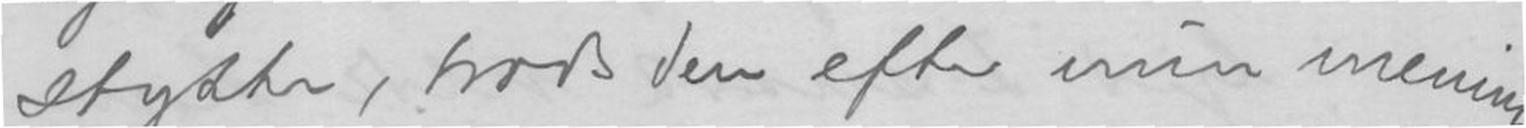stykke,trods den efter min mening
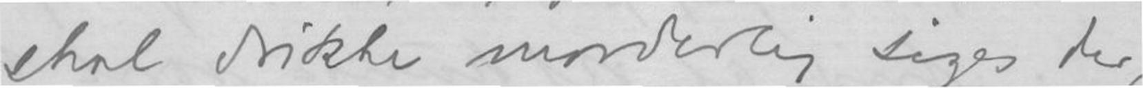skal drikke morderlig siges der,
